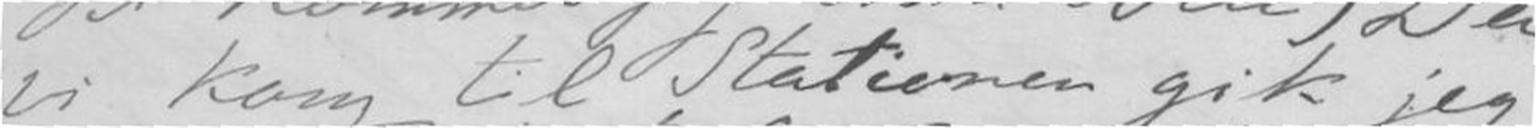vi kom til Stationen gik jeg
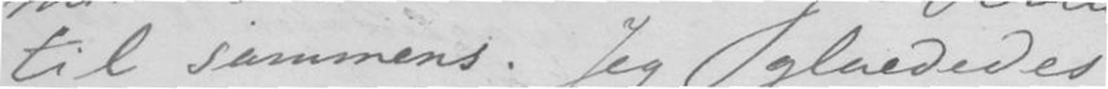til sammens. Jeg glaededes
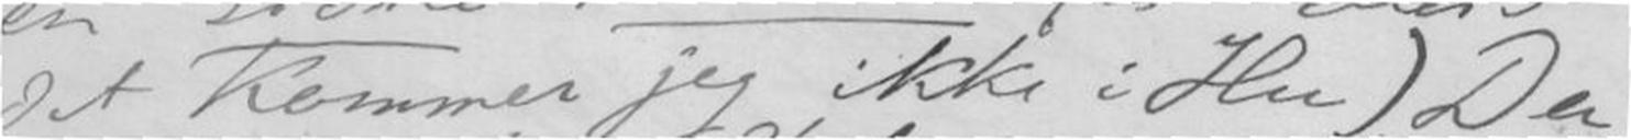det kommer jeg ikke i Hu) Da
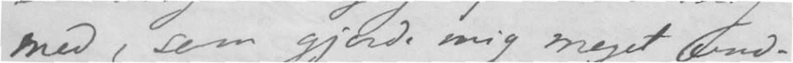med, som gjorde mig meget und-
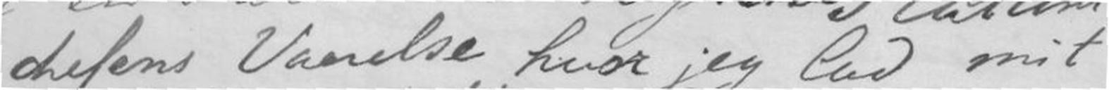chefens Vaerelse hvor jeg lod mit
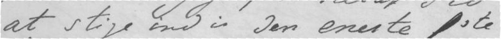at stige ind i den eneste 1ste
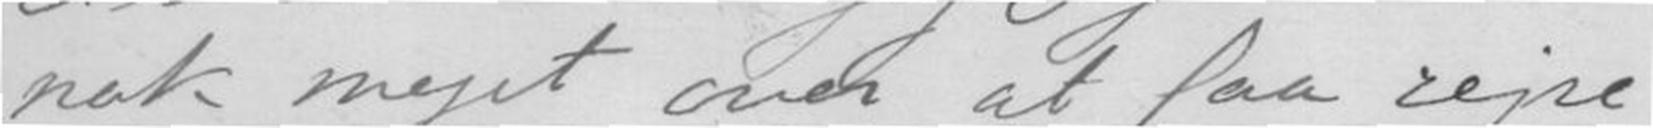nok meget over at faa rejse
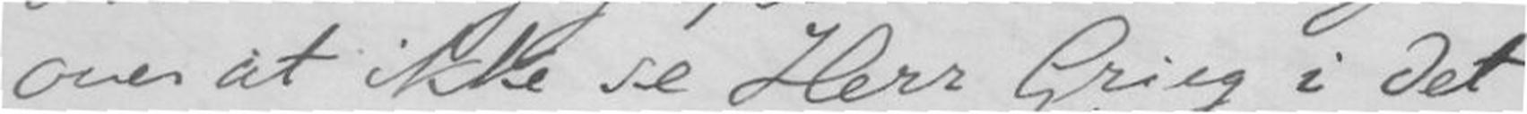over at ikke se Herr Grieg i Det
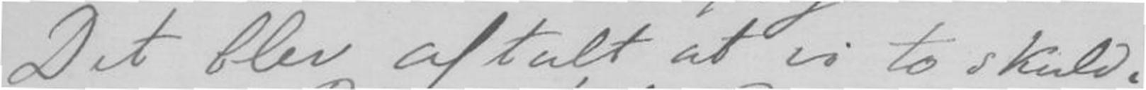Det blir aftalt at vi to skulde
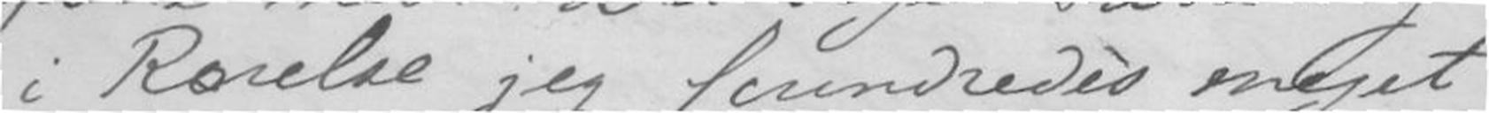i Rørelse jeg forundredés meget
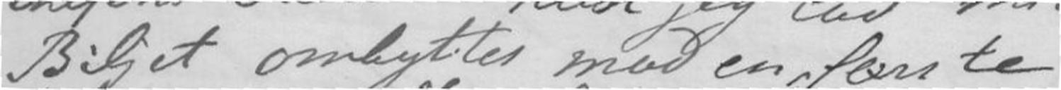Biljet ombyttes mod en første
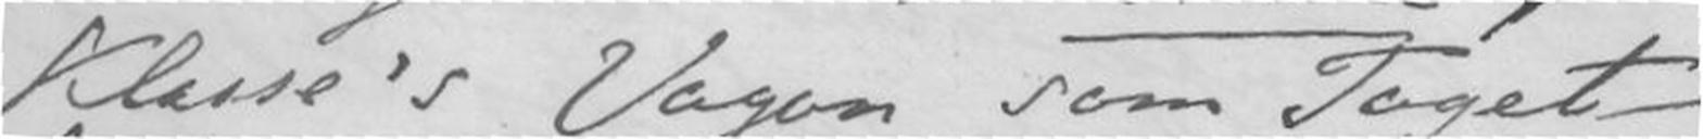Klasse's Vagon som Toget-
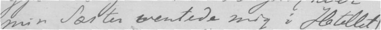min Søster ventede mig i Hotellet)
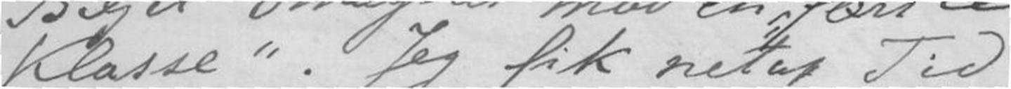Klasse". Jeg fik netop Tid
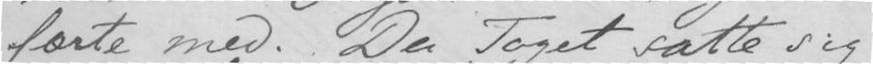førte med. Da Toget satte sig
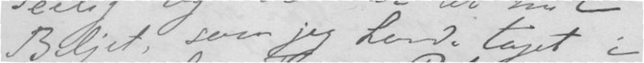Biljet, som jeg havde taget i
